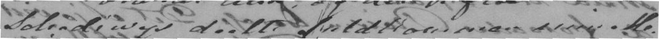Schediwys deelte fuldkommen min Me-
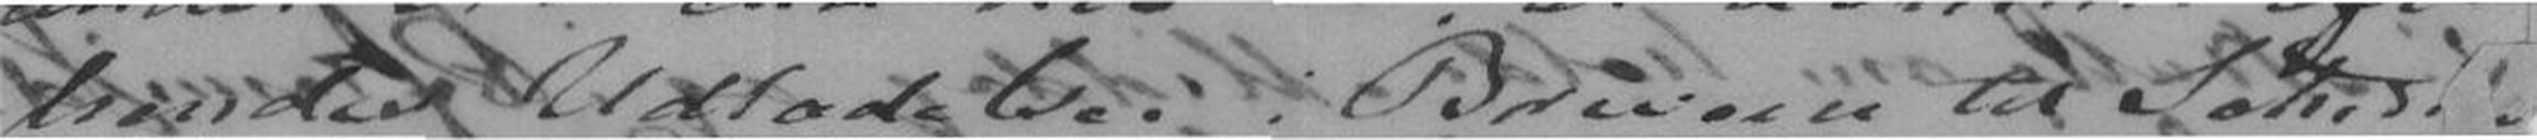hendes Udladelse i Brevene til Sched
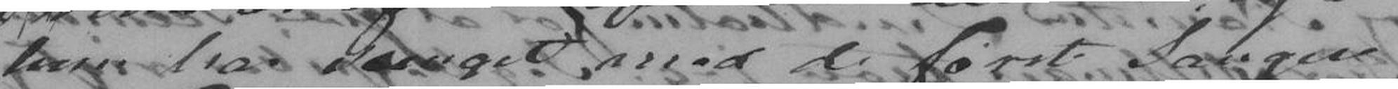hun har sunget med de første Sangere
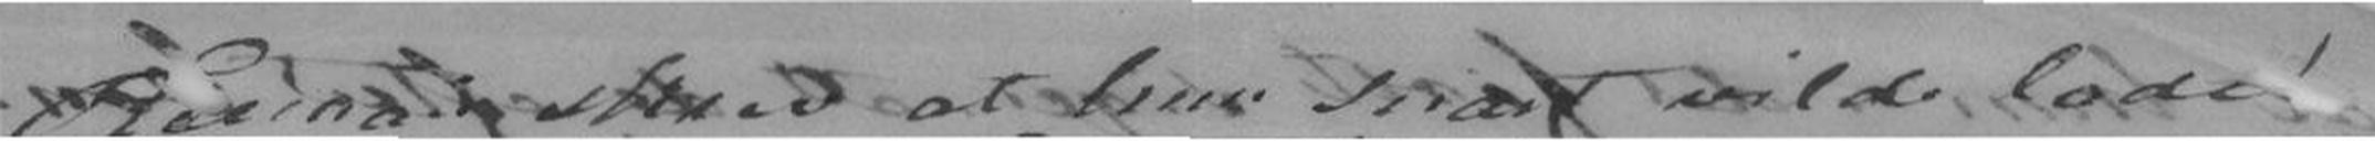Herman skrev at hun snart vilde lade!
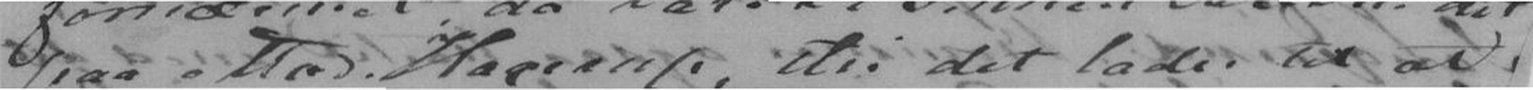paa Mad. Hagerup, thi det lader til at
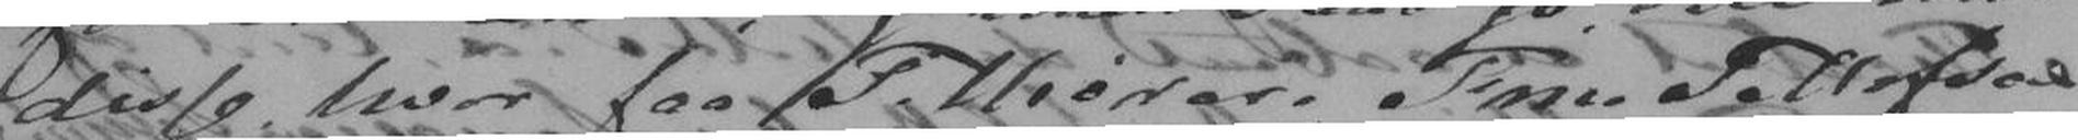disse hvor faa Tilhørere Frue Tellefsen
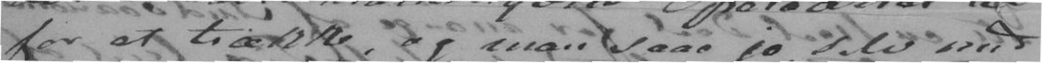for at trække, og man saae jo selv med
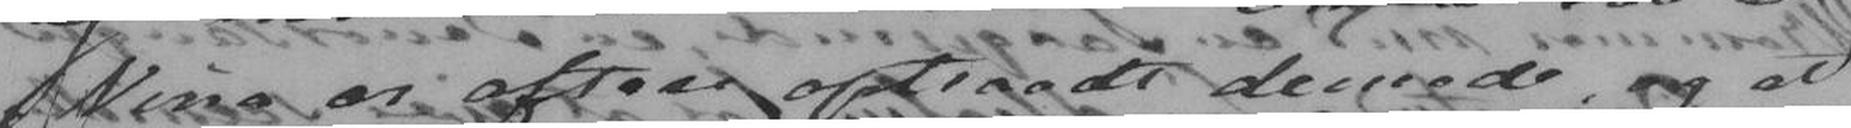Nina ei oftere optraadt dernede, og at
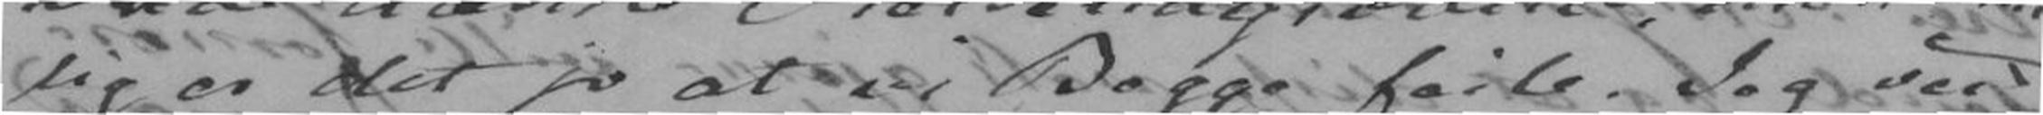lig er det jo at vi Begge feile. Jeg veed
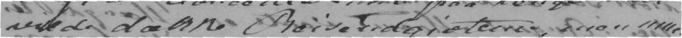vilde dække Reiseudgiftene, men mue-
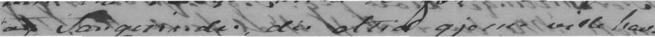og Sangerinder, der altid gjerne ville have
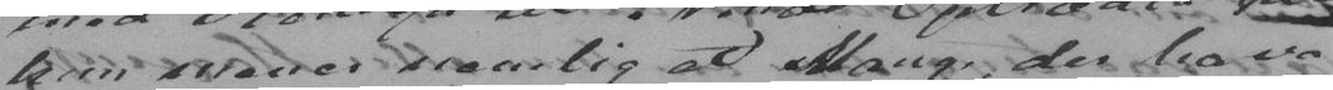hun mener nemlig at Mange der have
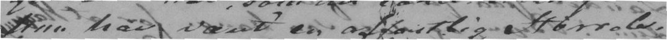hun har været en offentlig Størrelse
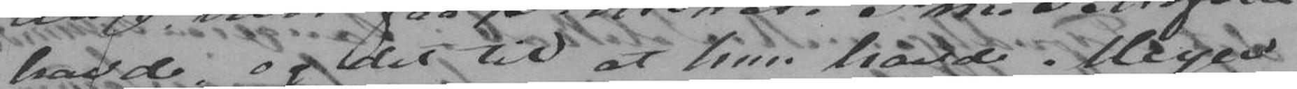havde, og det til at hun havde Meyer
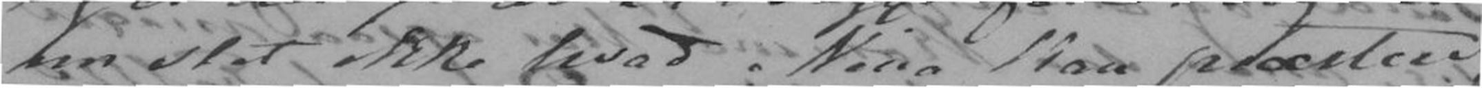nu slet ikke hvad Nina kan præstere,
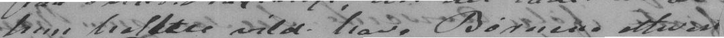hun hellere vilde have Børnene ethver
